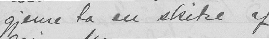gjerne ta en skitse af
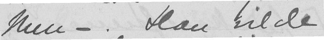Men - . Han vilde
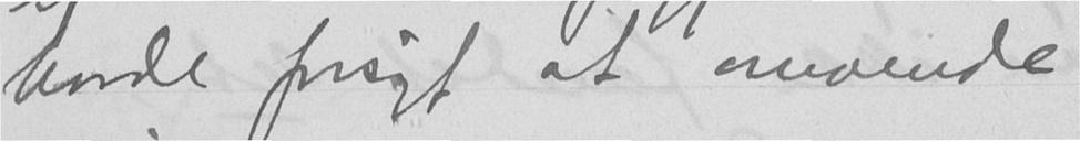havde forsøgt at omvende
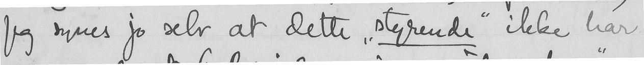Jeg synes jo selv at dette "styrende" ikke har
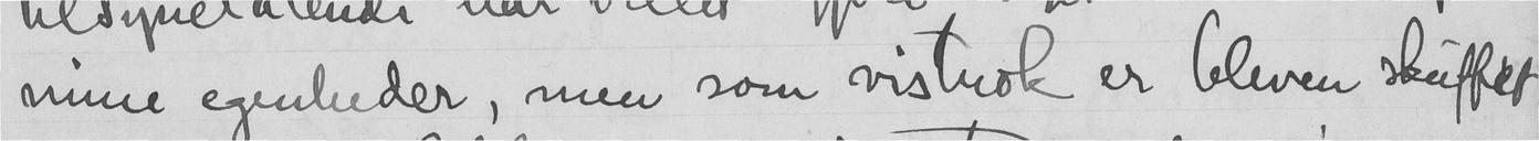mine egenheder, men som vistnok er bleven skuffet
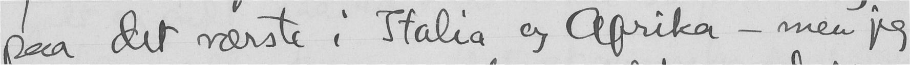paa det værste i Italia og Afrika, - men jeg
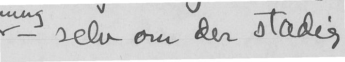- selv om der stadig
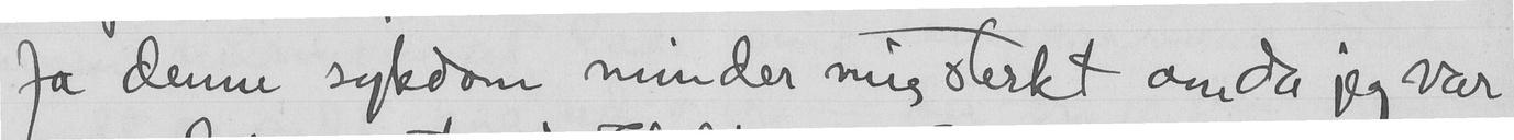Ja denne sykdom minder mig sterkt om da jeg var
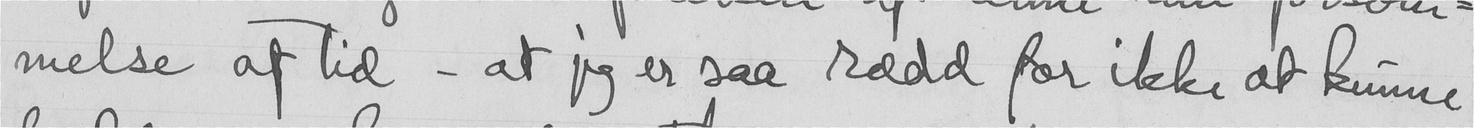melse af tid - at jeg er saa rædd for ikke at kunne
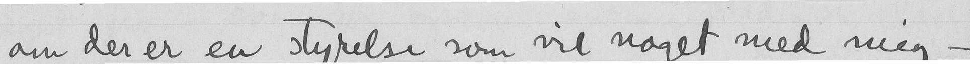om der er en styrelse som vil noget med mig -
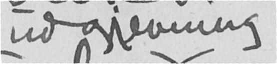udgjevning
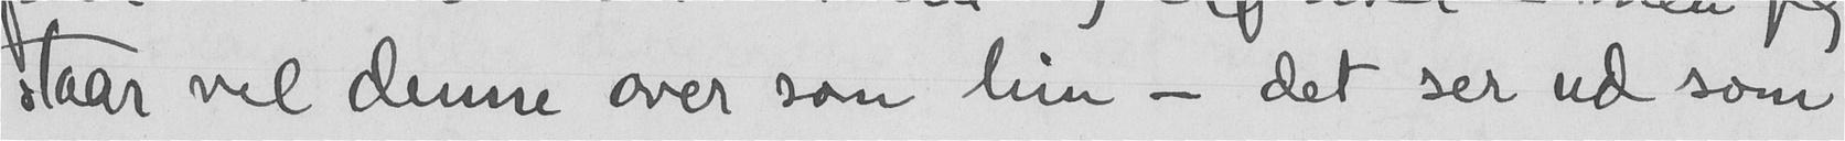staar vel denne over som hin - det ser ud som
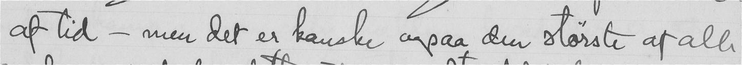af tid - men det er kanske ogsaa den største af alle
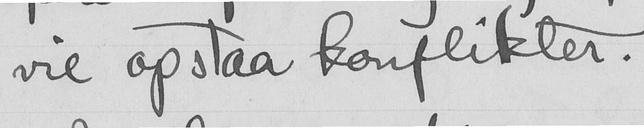vil opstaa konflikter.
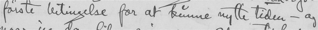første betingelse for at kunne nytte tiden - og
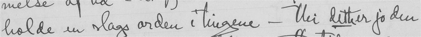holde en slags orden i tingene - thi dette er jo den
